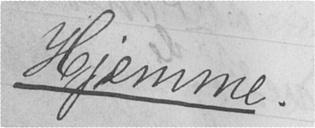Hjemme.
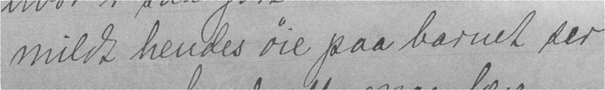mildt hendes øie paa barnet ser
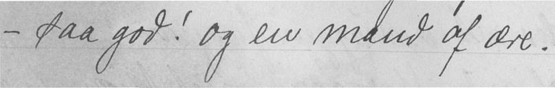- saa god! og en mand af ære.
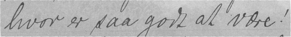hvor er saa godt at være!
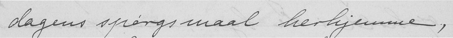dagens spørgsmaal herhjemme,
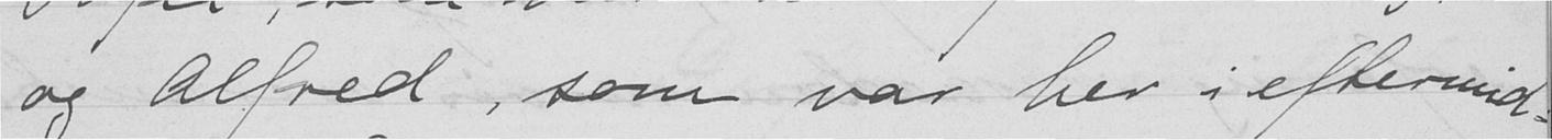og Alfred, som var her i eftermid-
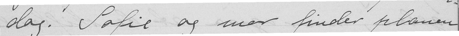dag. Sofie og mor finder planen
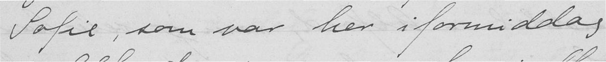Sofie, som var her i formiddag,
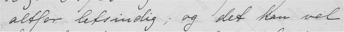altfor letsindig; og det kan vel
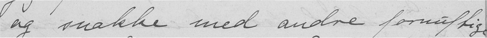og snakke med andre fornuftige
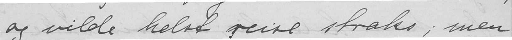og vilde helst reise straks; men
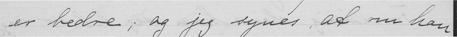er bedre; og jeg synes, at om han
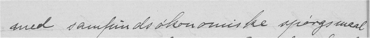med samfundsøkonomiske spørgsmaal
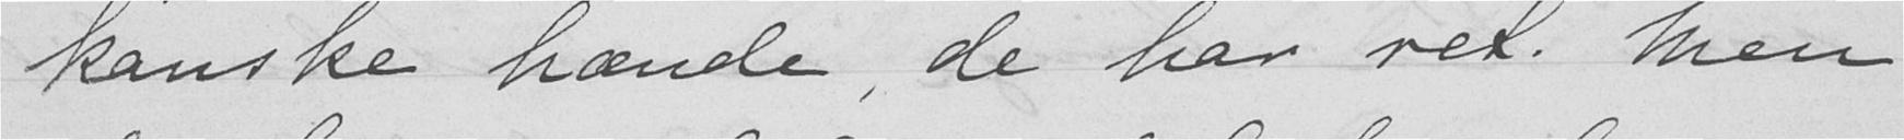kanske hænde, de har ret. Men
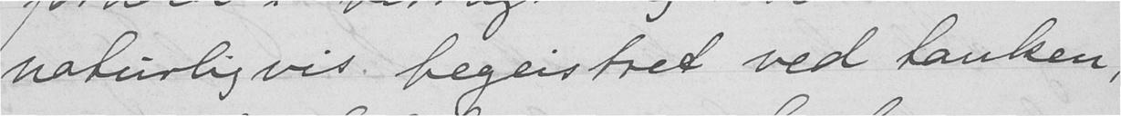naturligvis begeistret ved tanken,
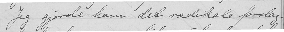Jeg gjorde ham det radikale forslag
